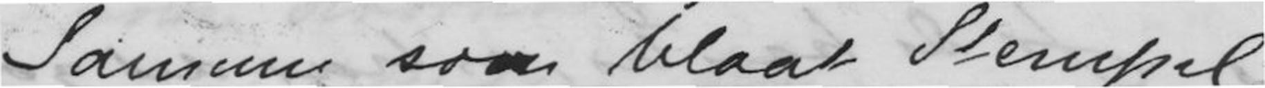samme som blaat Stempel
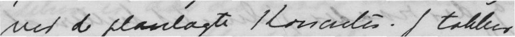ved de planlagte Koncerter. J takker
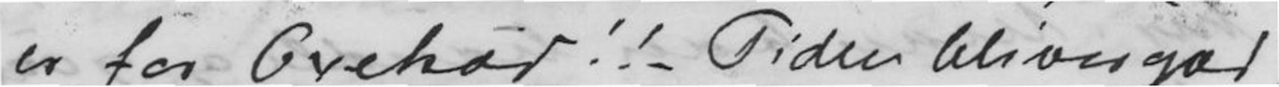er for Oxekød!! Tiden bliver god,
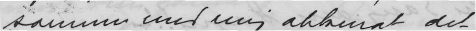sammen med mig akkurat det
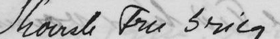Kaerste Fru Grieg!
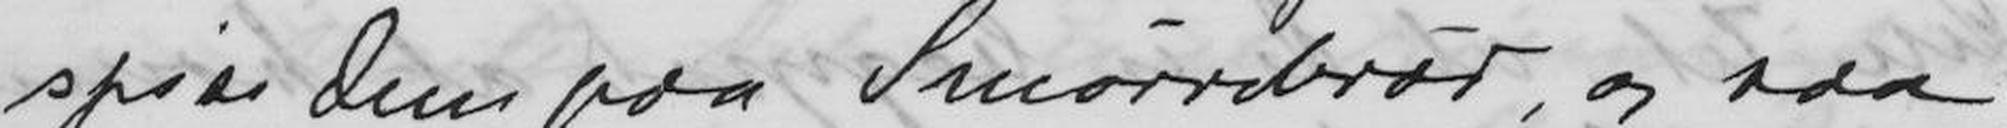spise Dem paa Smørrebrød og saa
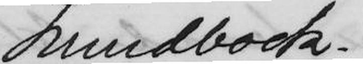Lundbaek.
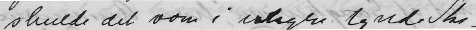skulde det vaere i tynde Ski-
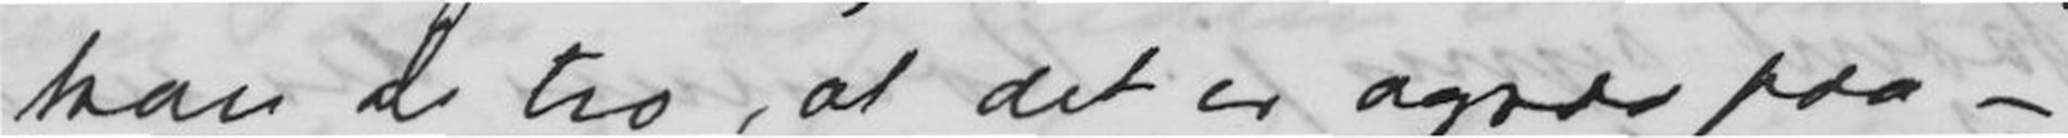kan d tro, at det er ogsaa paa-
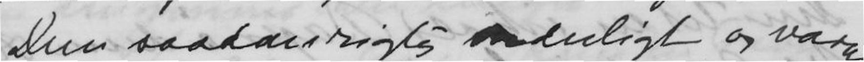Dem saadan rigtig inderligt og varmt
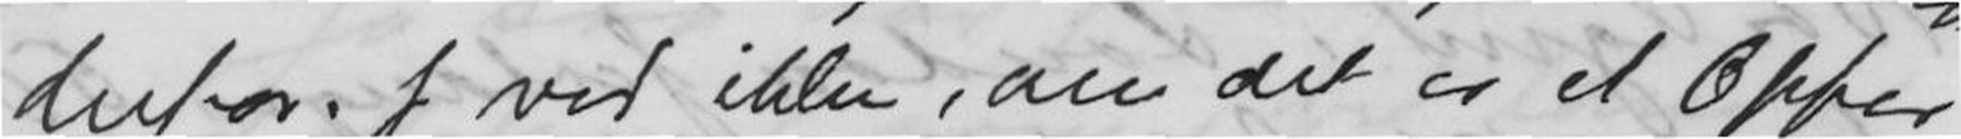derfor. J ved ikke, om de er et Offer
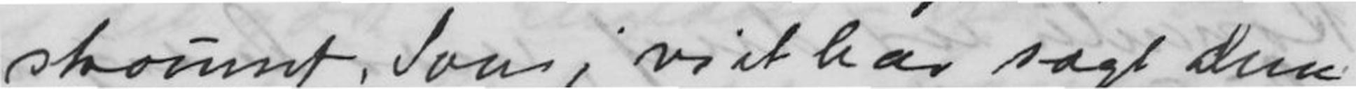skønnet. Som j vist har sagt Dem
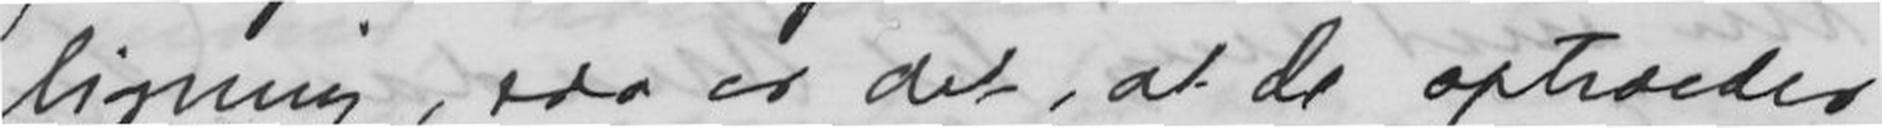ligning, saa er det, at De optraeder
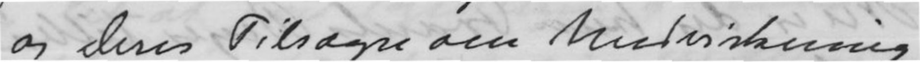og Deres Tilsagn om Medvirkning
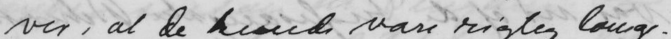ver, at De kunde vare rigtig laenge.
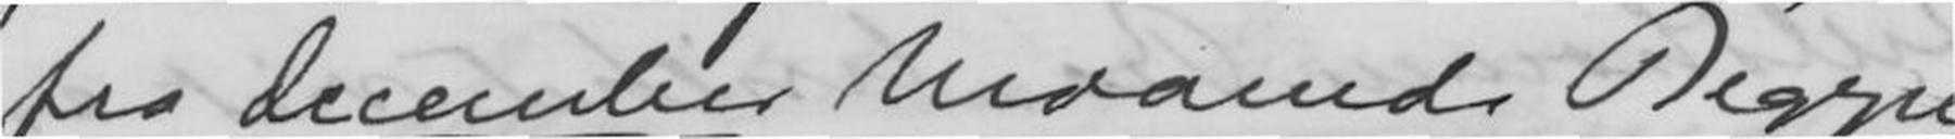fra December Maanede Begyndelse
